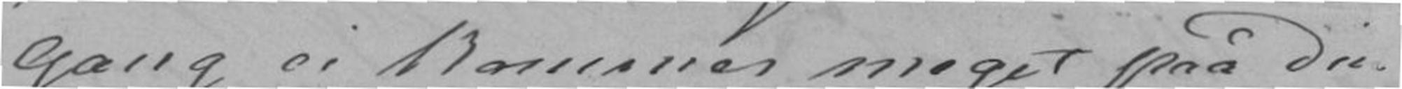Gang ei kommer meget paa Din
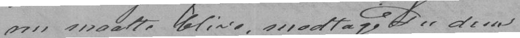nu maatte blive, modtage Du dens
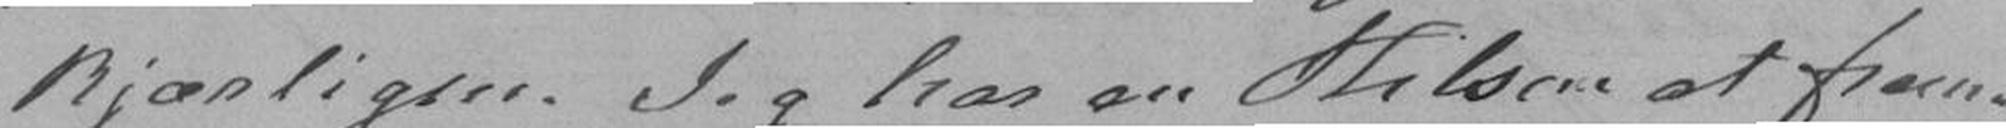kjærligen. Jeg har en Hilsen at frem-
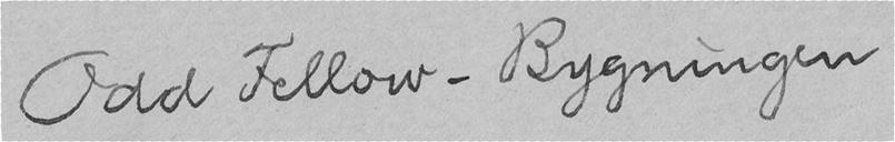Odd Fellow-Bygningen
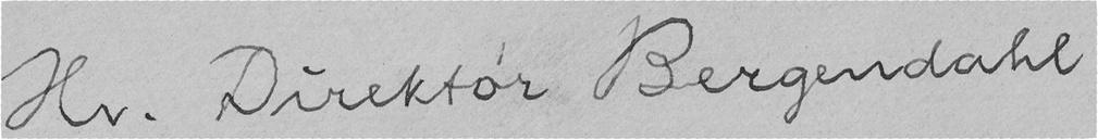Hr. Direktør Bergendahl
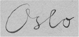Oslo
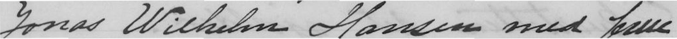Jonas Wilhelm Hansen med frue
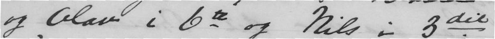og Olav i 6te og Nils i 3die
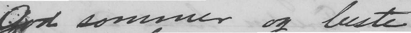God sommer og beste
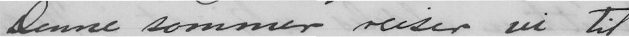Denne sommer reiser vi til
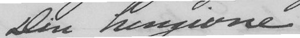Din hengivne
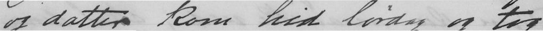og datter kom hid lørdag og tog
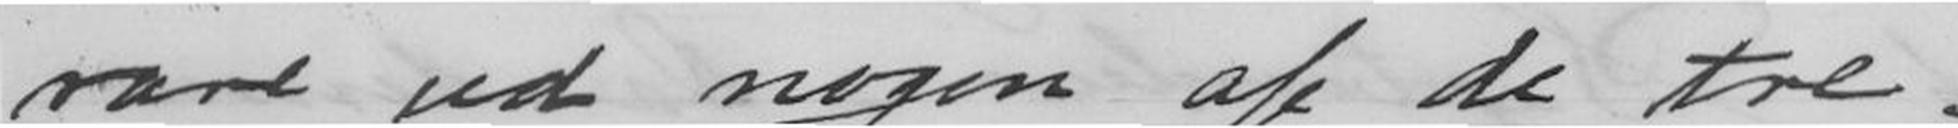rare ud nogen af de tre.
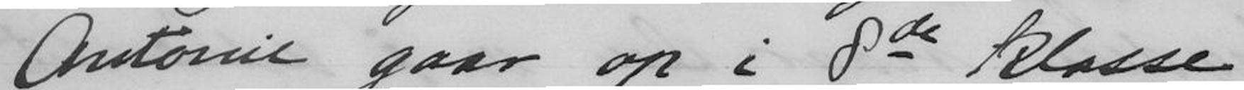Antonie gaar op i 8de klasse
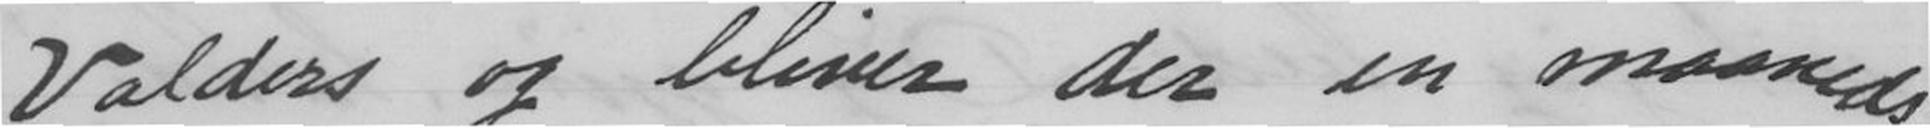Valders og bliver der en maaneds
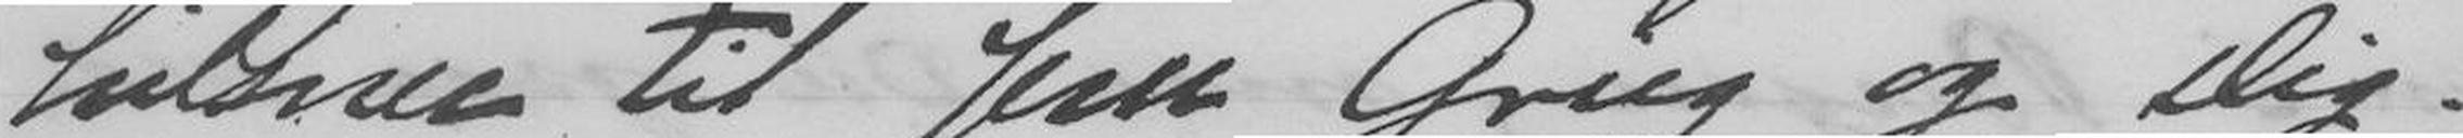hilsner til fru Grieg og Dig.
